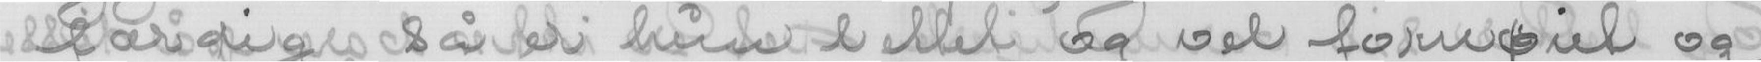færdig, så er hun lettet og vel fornøiet og
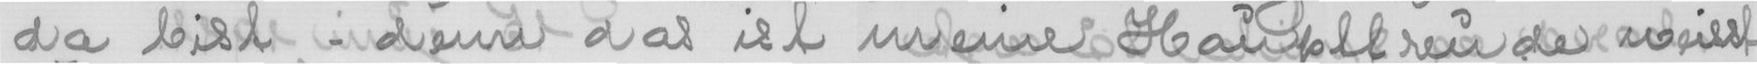da bist - denn das ist meine Hauptfreude weisst
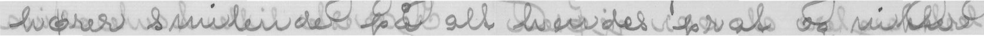hører smilende på alt hendes prat og nikker
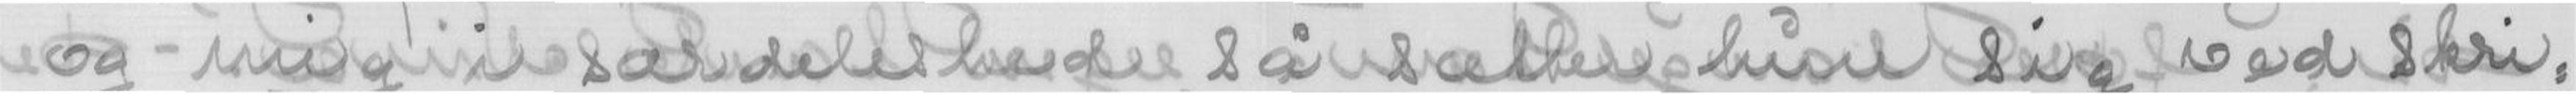og mig i særdeleshed, så sætter hun sig ved skri-
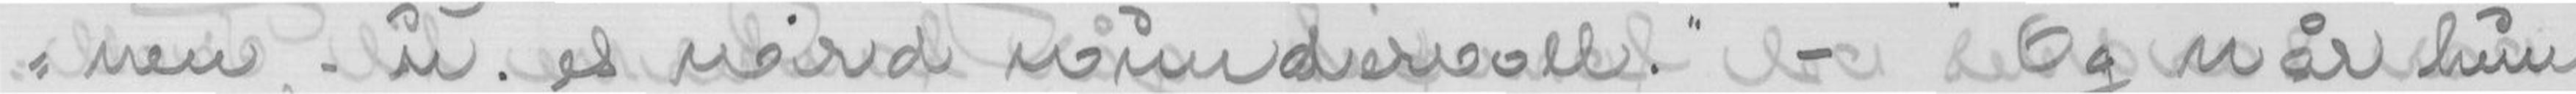nen, - u. es wird wundervoll." - Og når hun
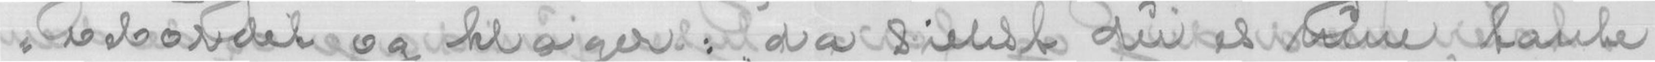vebordet og klager: "da siehst du es nun, tante
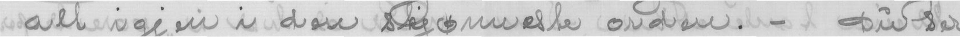alt igjen i den skjønneste orden. - Du ser
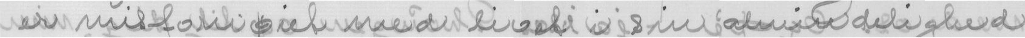er misfornøiet med livet i sin almindelighed
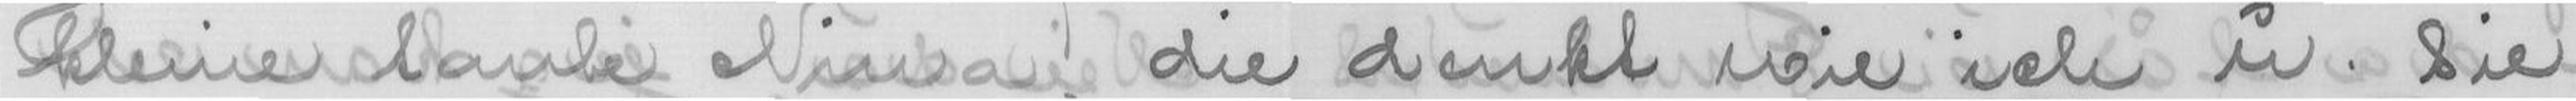kleine tante Nina, die denkt wie ich u. sie
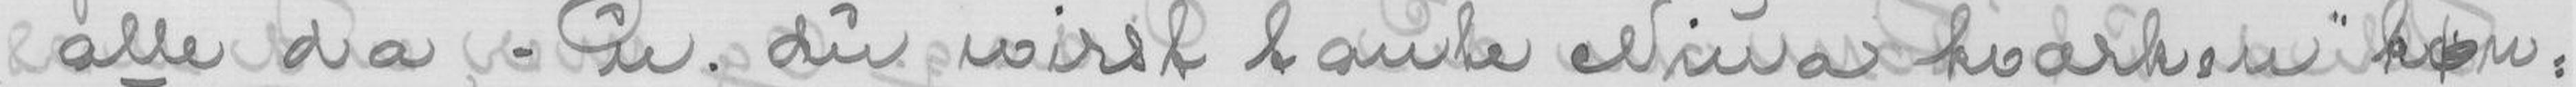alle da, - u du wirst tante Nina "kværken" køn-
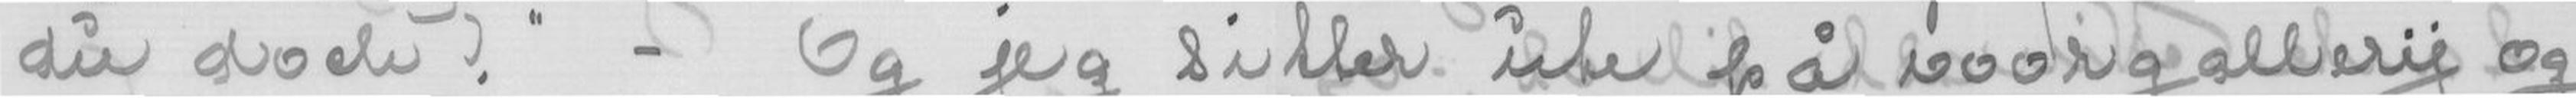du doch!" - Og jeg sitter ute på voorgallery og
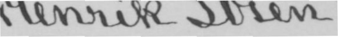Henrik Ibsen.
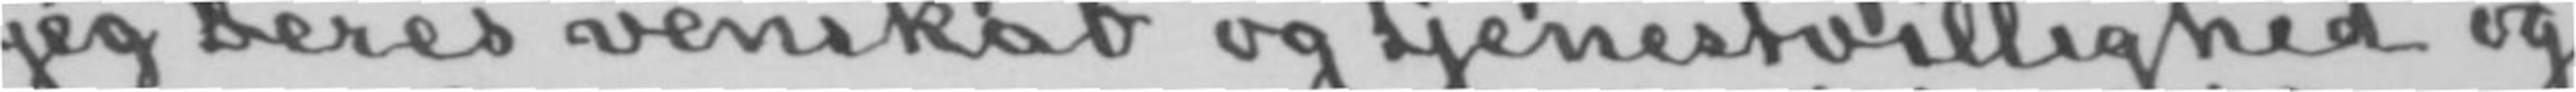jeg Deres venskab og tjenestvillighed og
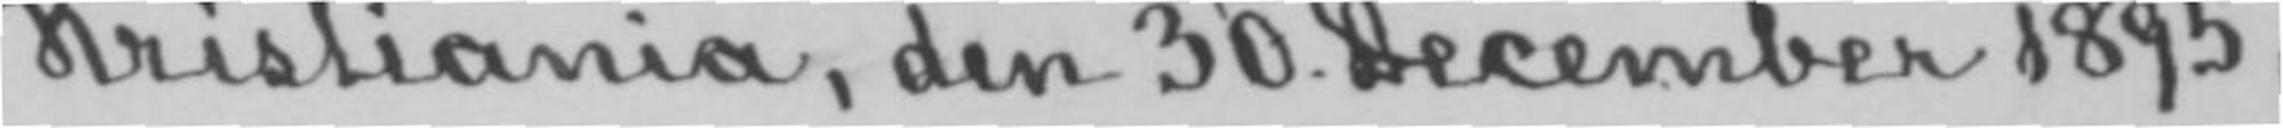Kristiania, den 30. December 1895.
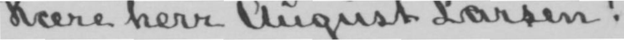Kære herr August Larsen!
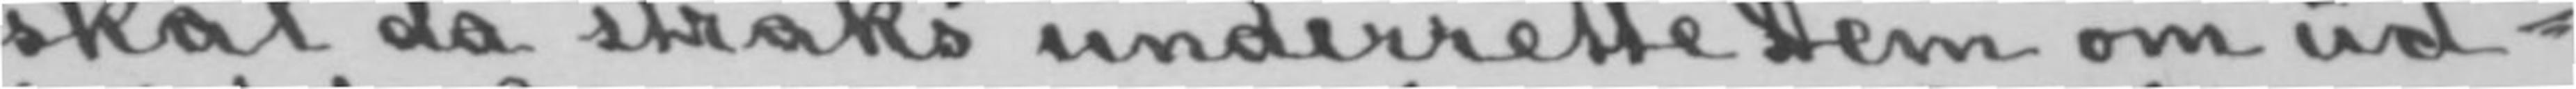skal da straks underrette Dem om ud-
